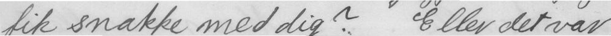fik snakke med dig? Eller det var
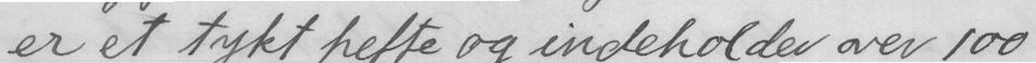er et tykt hefte og indeholder over 100
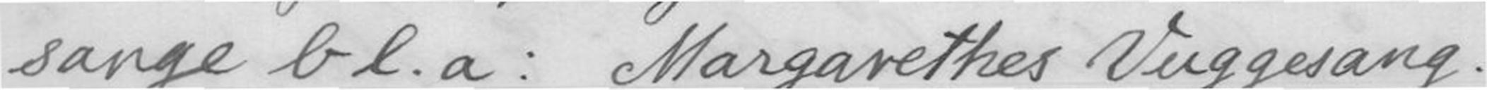sange b l.a: Margarethes Vuggesang.
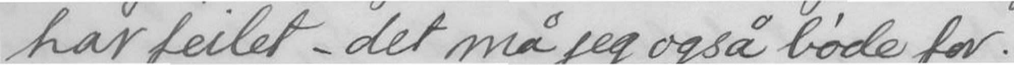har feilet - det må jeg også bøde for.
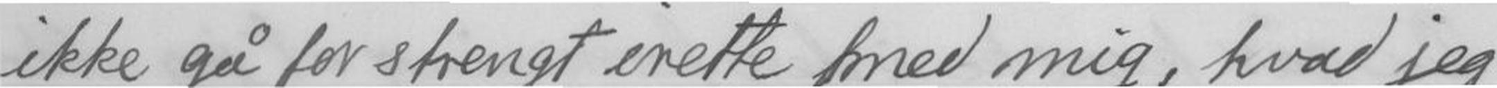ikke gå strengt irette med mig, hvad jeg
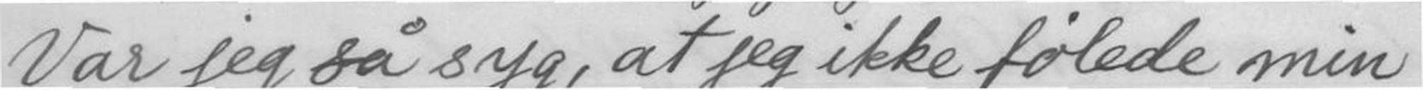Var jeg så syg, at jeg ikke følede min
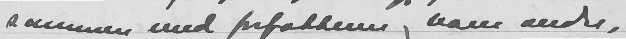sammen med forfatteren og noen andre,
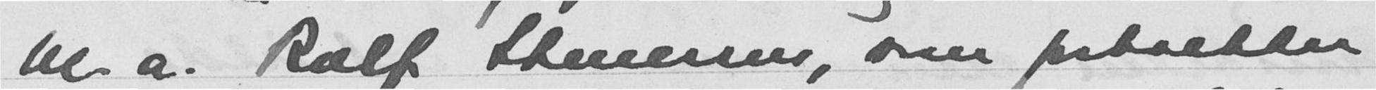bl. a. Rolf Stenersen, som fortsetter
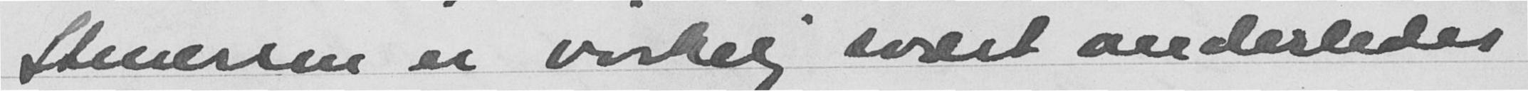Stenersen er virkelig svært anderledes
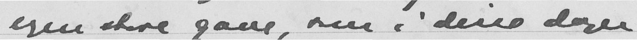egen store gave, som i disse dager
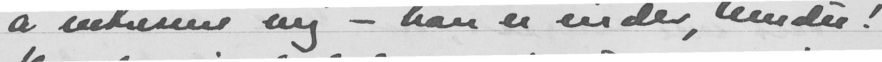å interessere mig - han er inder, hindu!
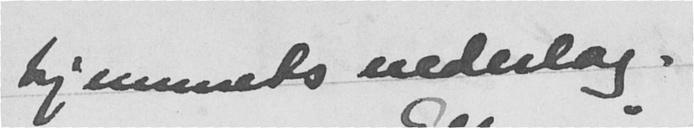hjemmets nederlag.
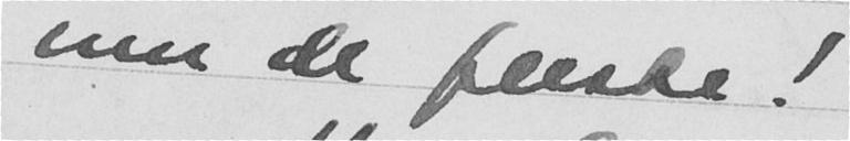enn de fleste.
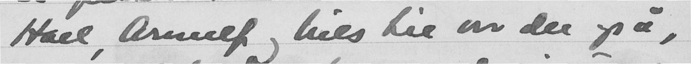Hoel, Arnulf og Nils Lie var der også,
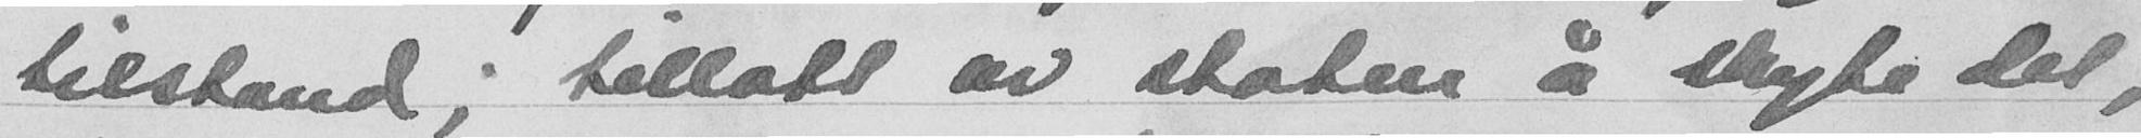tilstand; tillatt av staten å skyte det,
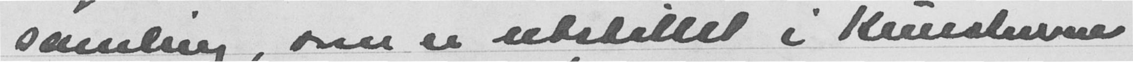samling, som er utstillet i Kunstnernes
